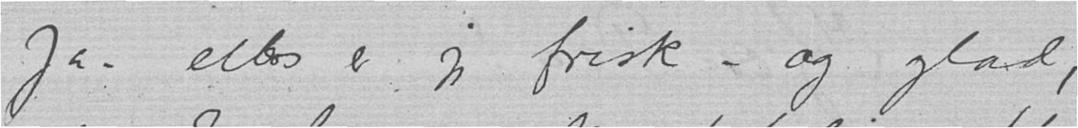Ja - ellers er jeg frisk - og glad,
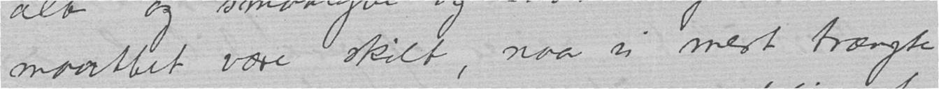maattet være skilt, naar vi mest trængte
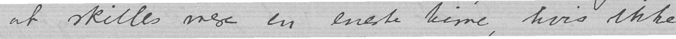at skilles mer en eneste time, hvis ikke
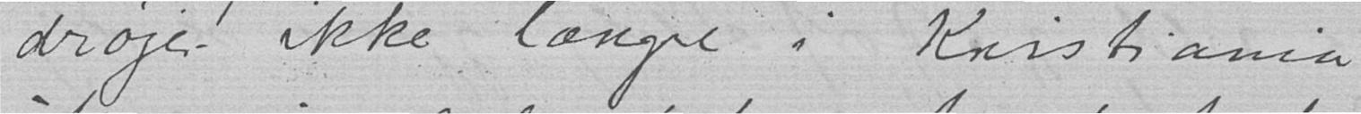drøjer ikke længre i Kristiania
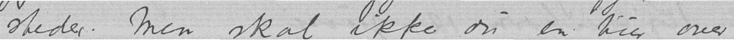steder. Men skal ikke du en tur over
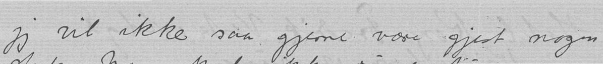jeg vil ikke saa gjerne være gjest nogen
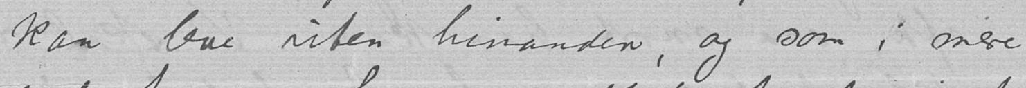kan leve uten hinanden, og som i mere
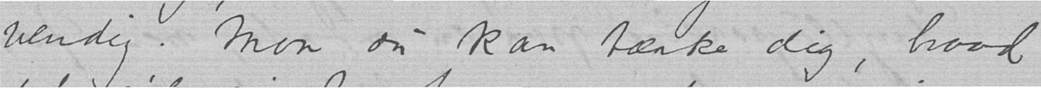vendig. Mon du kan tænke dig, hvad
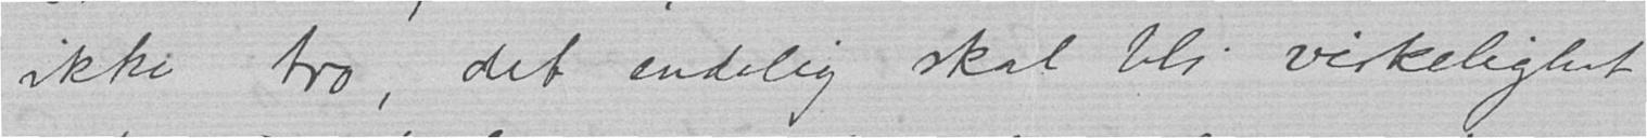ikke tro, det endelig skal bli virkelighet
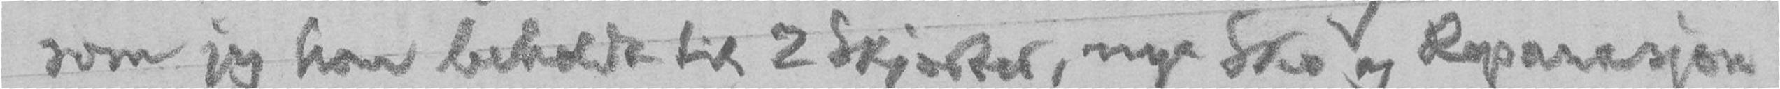som jeg har beholdt til 2 Skjorter, nye Sko og Reparasjon
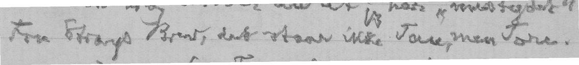Fru Strays Brev, det staar ikke Tau, men Tore.
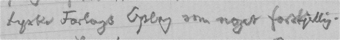tyske Forlags Oplag som noget forskjellig.
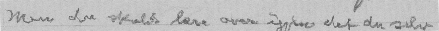Men du skulde læse over igjen det du selv
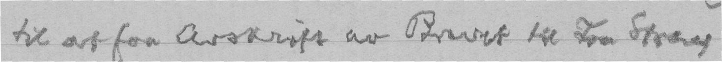til at faa Avskrift av Brevet til Fru Stray
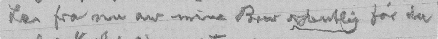Læs fra nu av mine Brev ordentlig før du
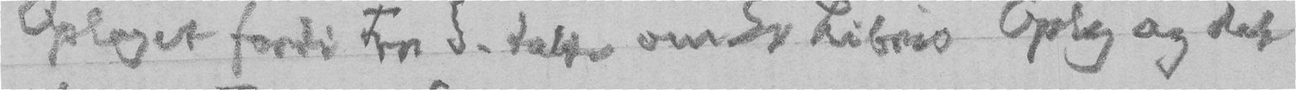Oplaget fordi Fru S. talte om Ex Libris Oplag og det
